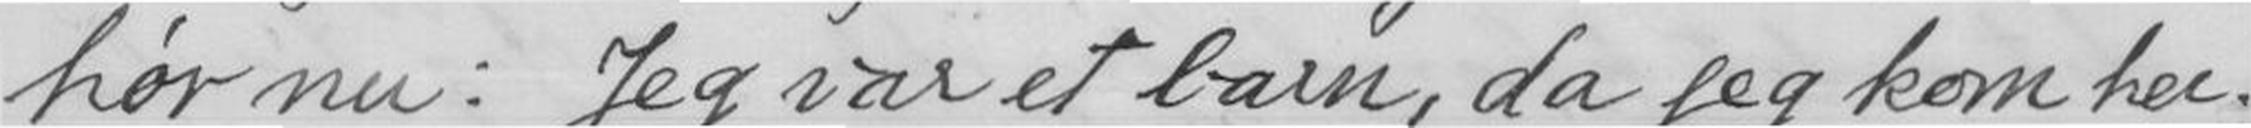hør nu: Jeg var et barn, da jeg kom her.
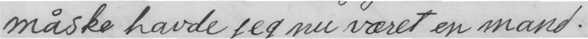måske havde jeg nu været en mand.
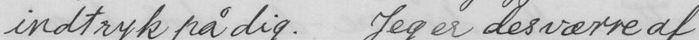indtryk på dig. Jeg er desværre af
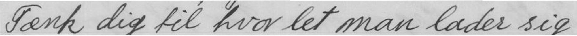Tænk dig til hvor let man lader sig
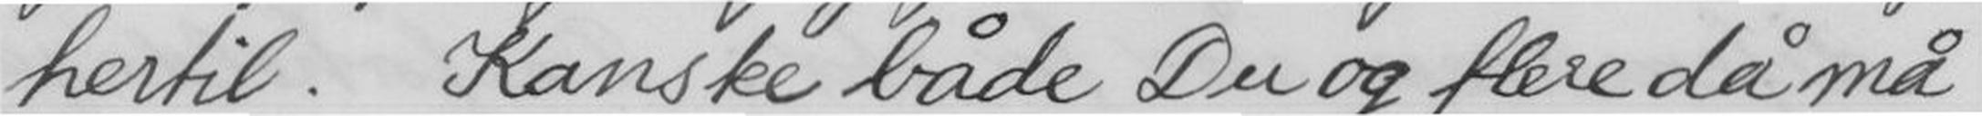hertil. Kanske både Du og flere då må
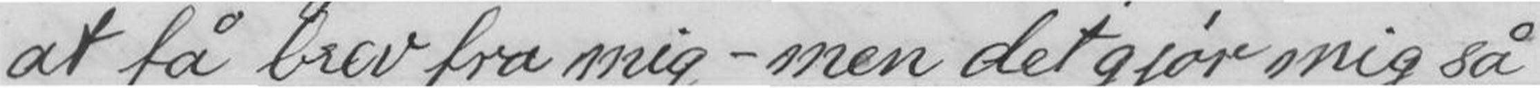at få brev fra mig - men det gjør mig så
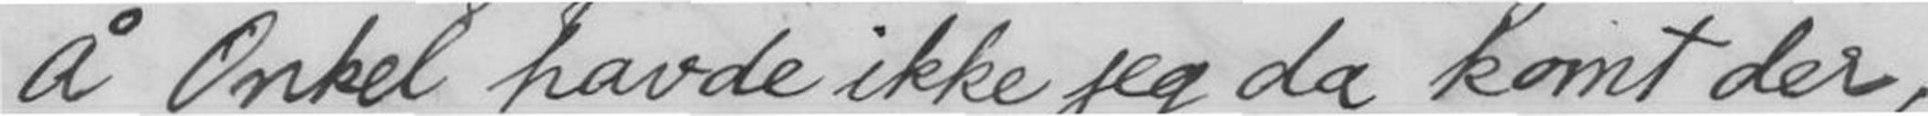Å Onkel havde ikke jeg da komt der,
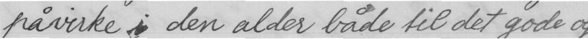påvirke i den alder både til det gode og
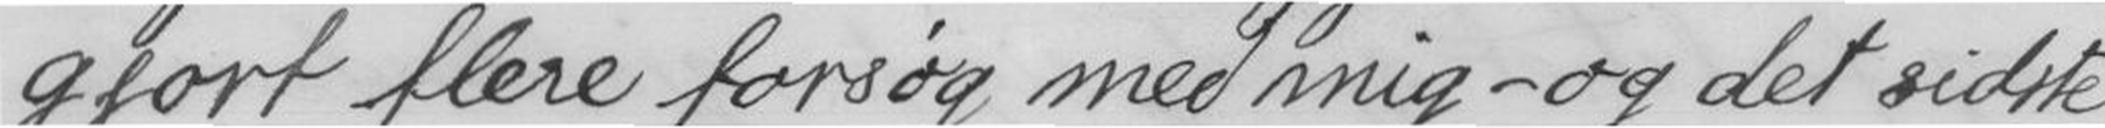gjort flere forsøg med mig - og det sidste
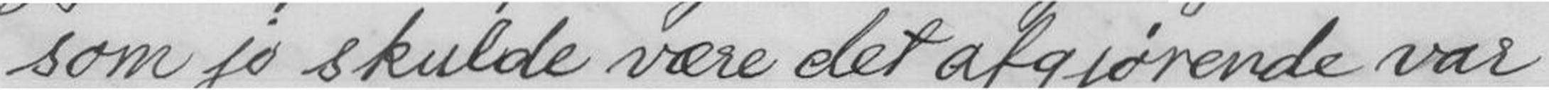som jo skulde være det afgjørende var
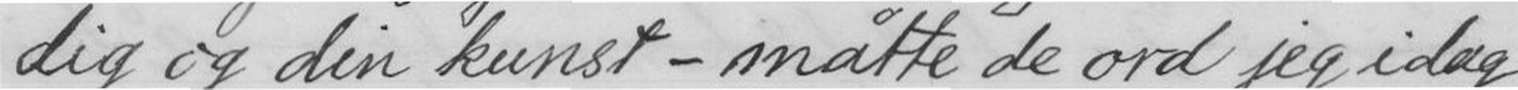dig og din kunst - måtte de ord jeg idag
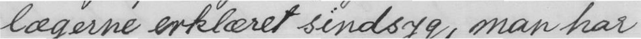lægerne erklæret sindsyg, man har
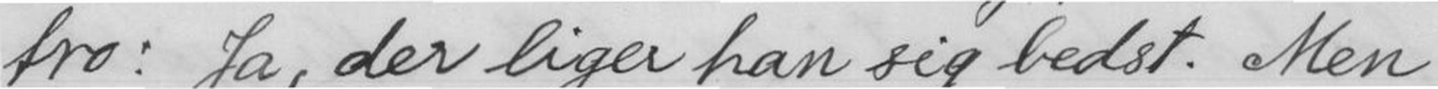tro: Ja, der liger han sig bedst. Men
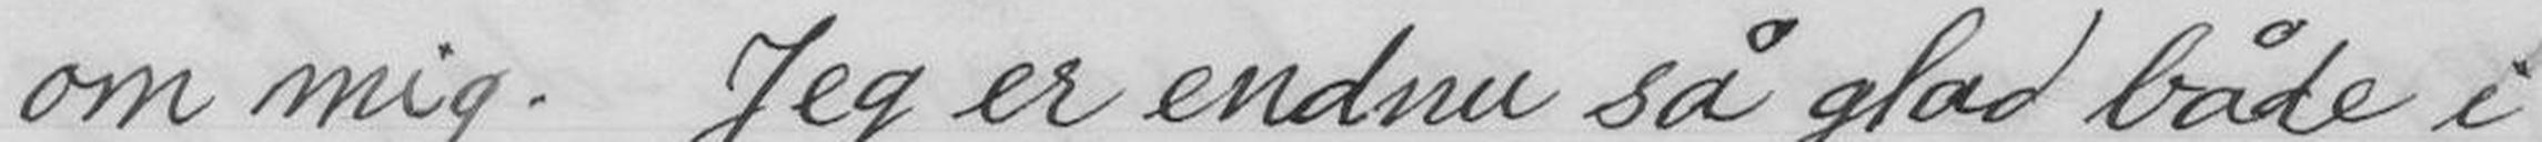om mig. Jeg er endnu så glad både i
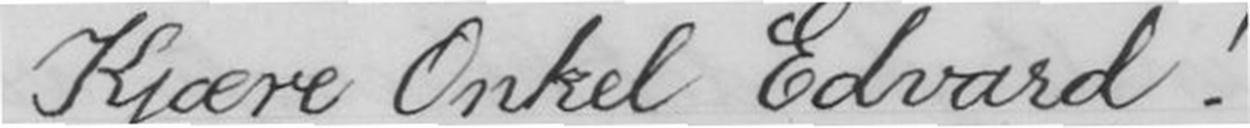Kjære Onkel Edvard!
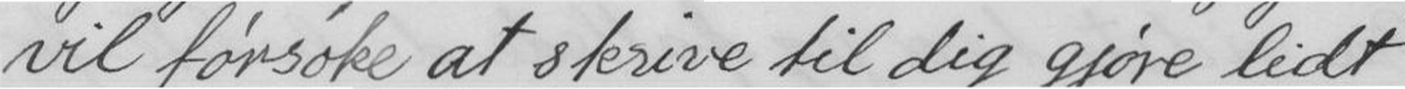vil forsøke at skrive til dig gjøre lidt
